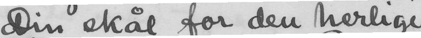Din skål for den herlige
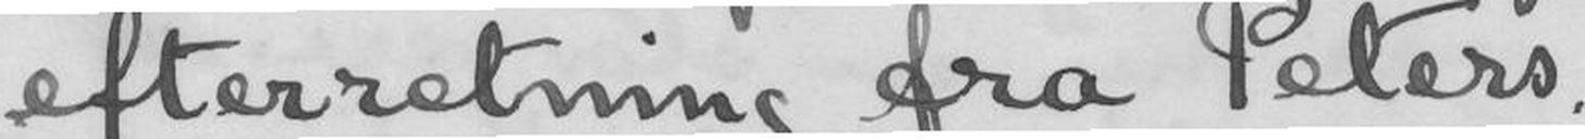efterretning fra Peters.
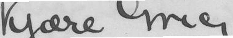Kjære Grieg!
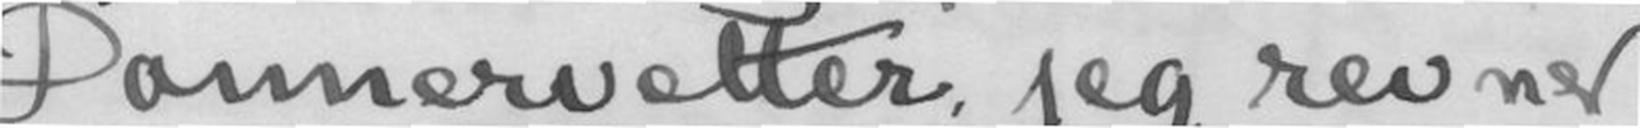Donnervetter, jeg revner
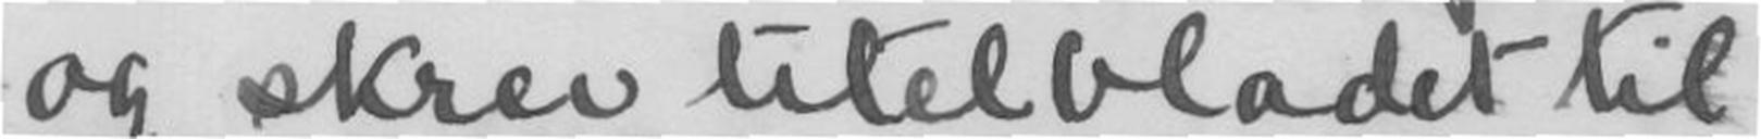og skrev titelbladet til
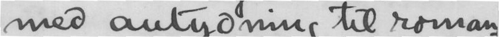med antydning til roman-
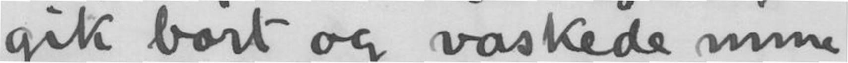gik bort og vaskede mine
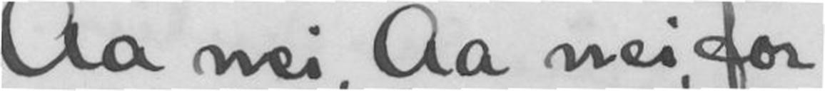Aa nei, Aa nei, for
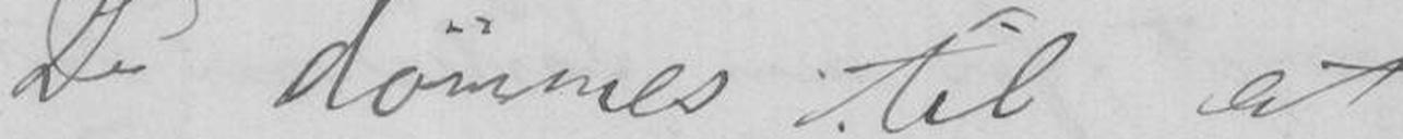Du dømmes til at
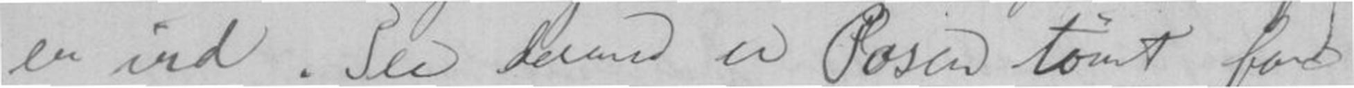en ind. See dermed er Posen tømt for
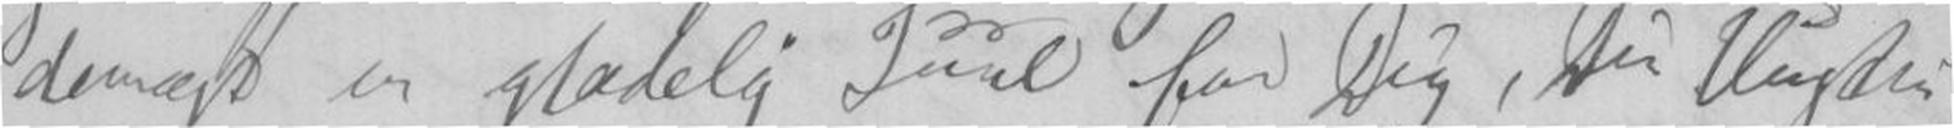dernæst en glædelig Juul for Dig, Din Hustru
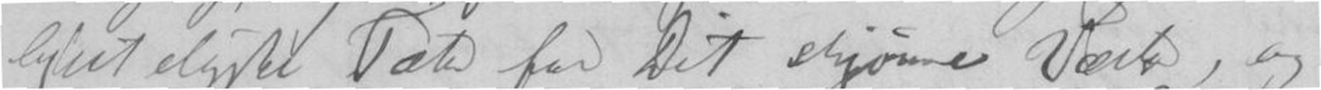lykeligste Tak for Dit skjønne Værk, og
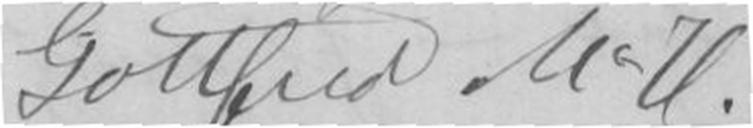Gottfred M=H.
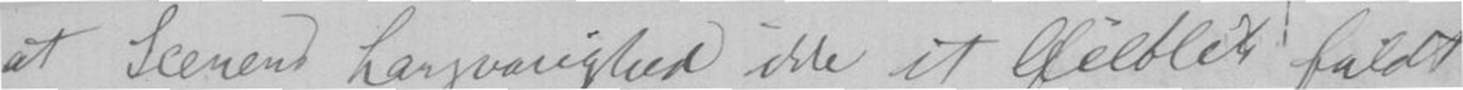at Scenens Langvarighed ikke et Øieblik faldt
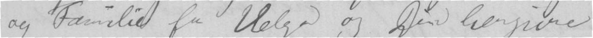og Familie fra Helga og Din hengivne
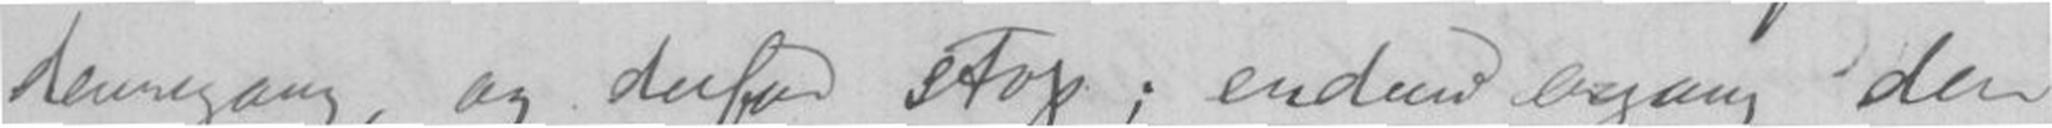dennegang, og derfor stop; endnu engang den
